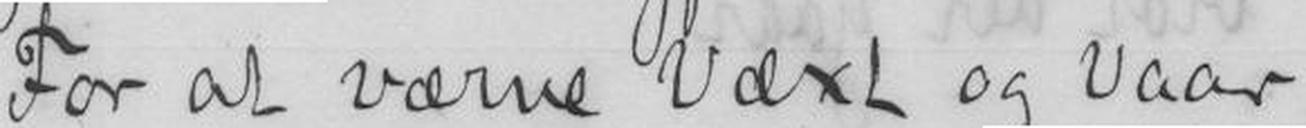For at værne væxt og Vaar
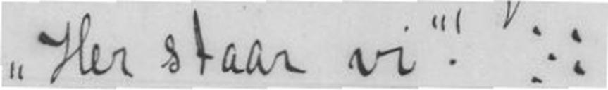Her staar vi! :/:
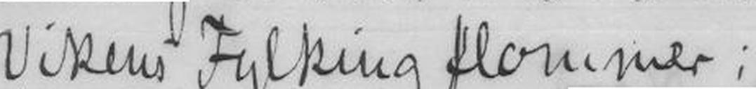Vikens Fylking flommer;
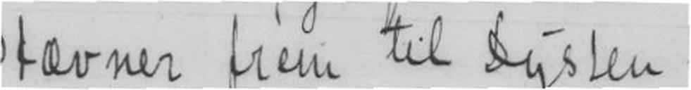stævner frem til Dysten.
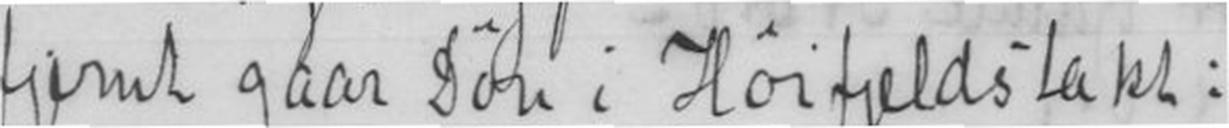fjernt gaar Døri i Høifjeldstakt:
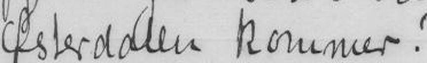Østerdalen kommer!
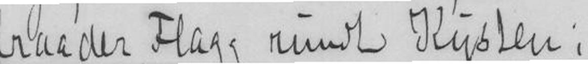fraader Flagg rundt Kysten;
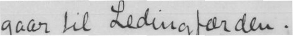gaar til Ledingfærden.
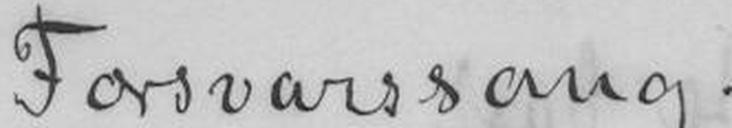Forsvarssang.
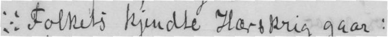:/:Folkets kjendte Hærkrig gaar:
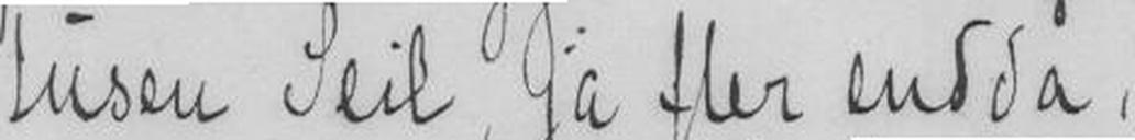tusen Seil, ja fler end da,
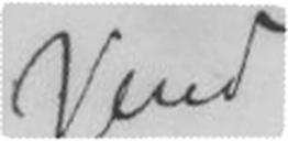Vend
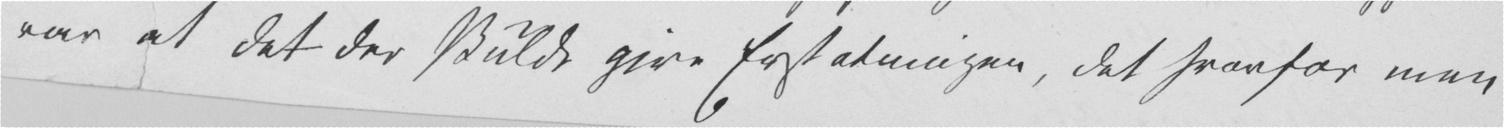var at det der skulde give Erstatningen, det hvorfor man
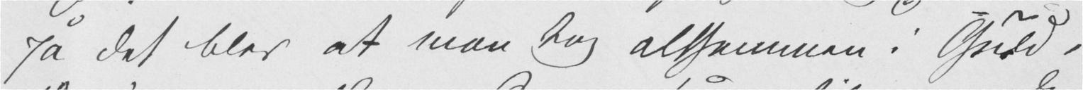på det blev at man tog altsammen i Guld,
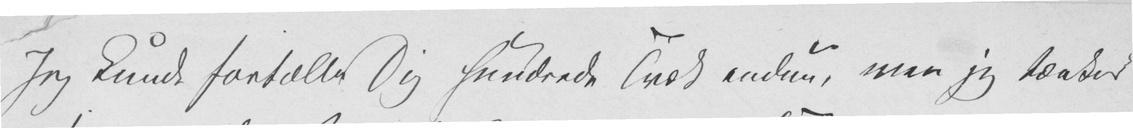Jeg kunde fortælle Dig hundrede Træk endnu, men jeg tænker
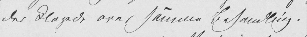der klagede over samme Behandling.
